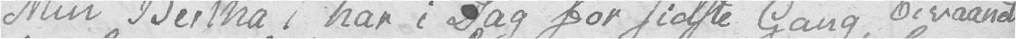Min Bertha! har i Dag for sidste Gang bivaanet
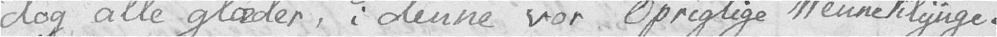dog alle glæder, i denne vor Oprigtige Venneklynge.
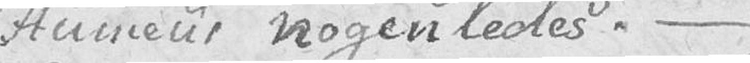Humeur nogenledes. –
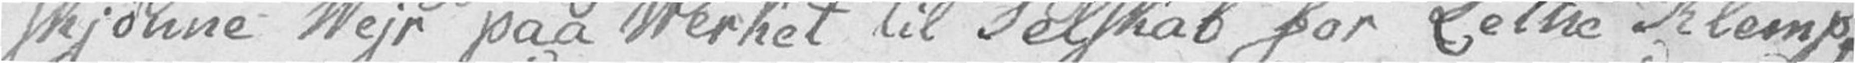skjønne Vejr paa Verket til Selskab for Lethe Klemp,
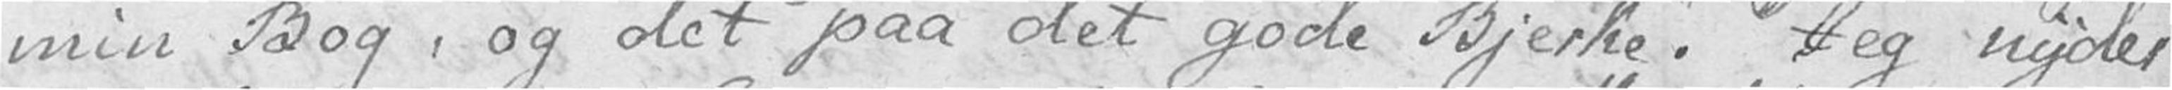min Bog, og det paa det gode Bjerke. Jeg nyder
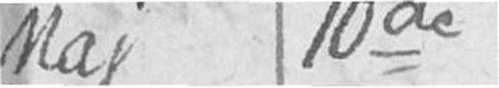Maj 10de
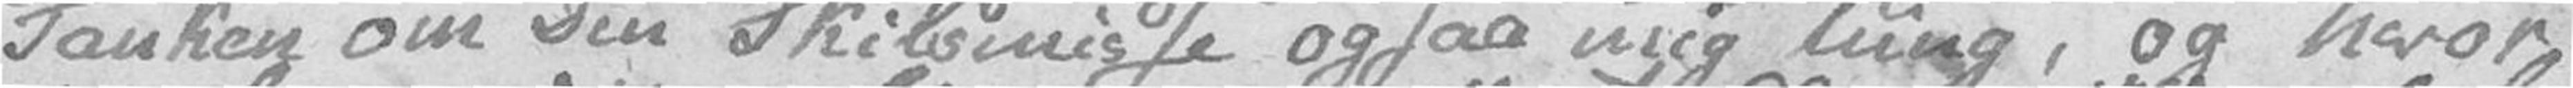Tanken om Din Skilsmisse ogsaa mig tung, og hvor
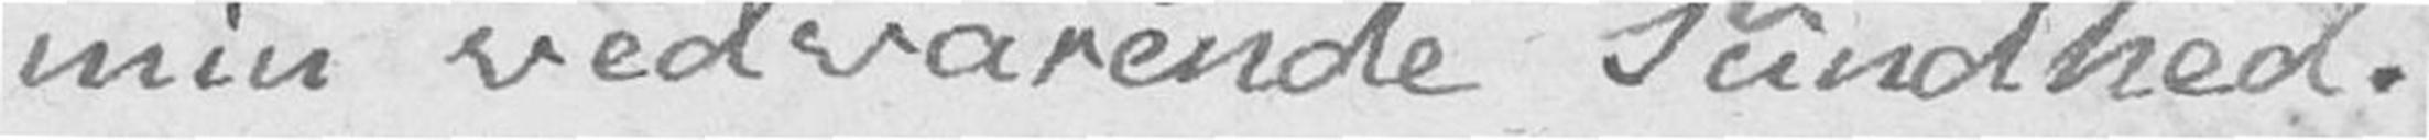min vedvarende Sundhed.
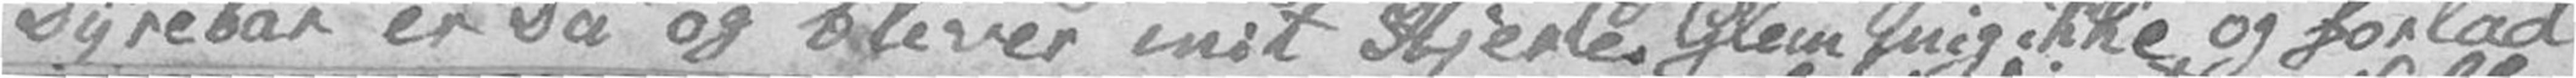Dyrebar er Du og bliver mit Hjerte. Glem mig ikke og forlad
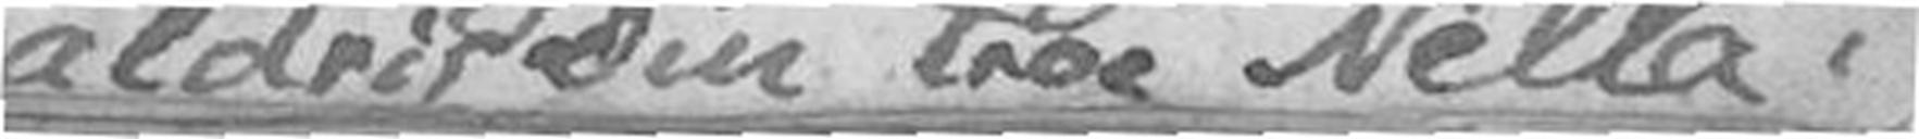aldrig Din troe Nella.
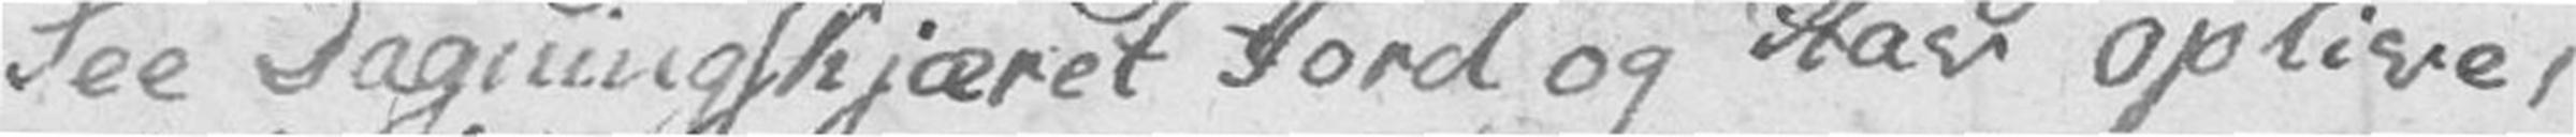See Dagningskjæret Jord og Hav opliver
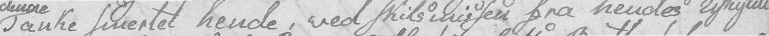denne Tanke smertet hende, ved skilsmissen fra hendes uskyldi
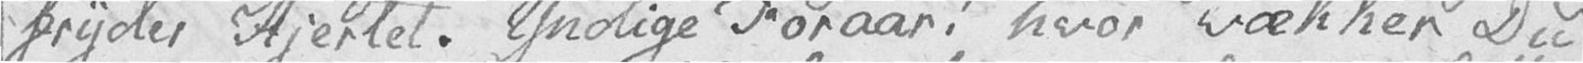fryder Hjertet. Yndige Foraar! hvor vækker Du
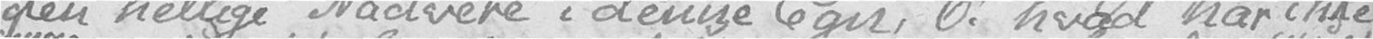den hellige Nadvere i denne Egn, O! hvad har ikke
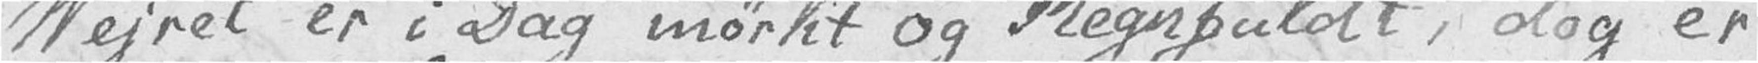Vejret er i Dag mørkt og Regnfuldt, dog er
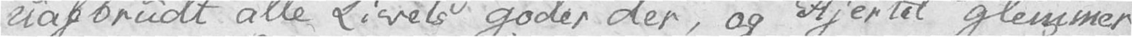uafbrudt alle Livets goder der, og Hjertet glemmer
